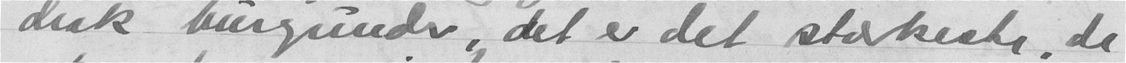drak burgunder, det er det stærkeste, de
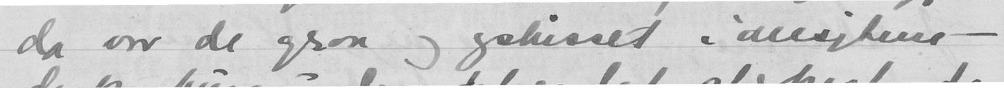da var de graa og ophisset i ansigtene -
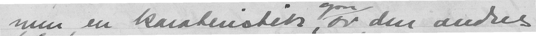men en karateristik av den andres
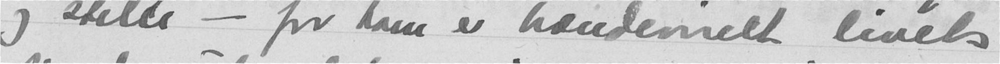og stille - for ham er brændevinet livets
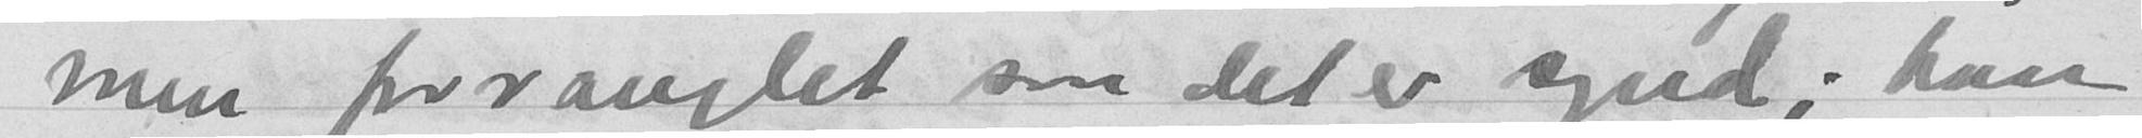men forranglet som det er synd; han
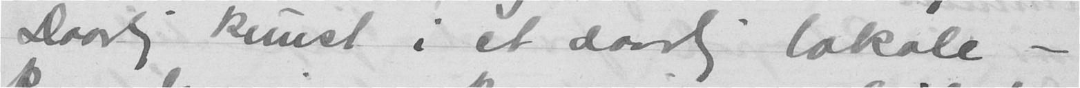Daarlig kunst i et daarlig lokale -
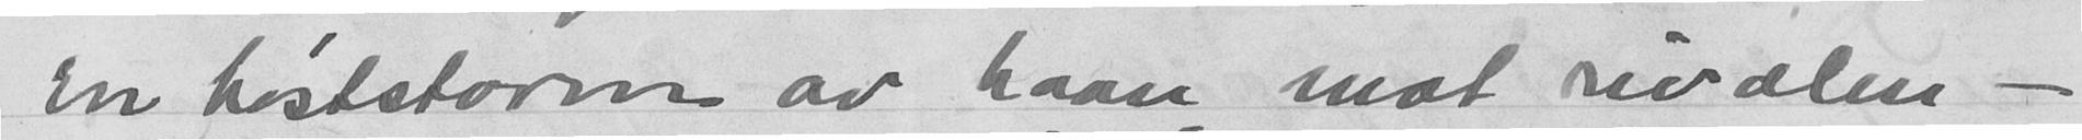En høststorm av haan mot rivalen -
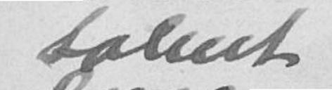talent
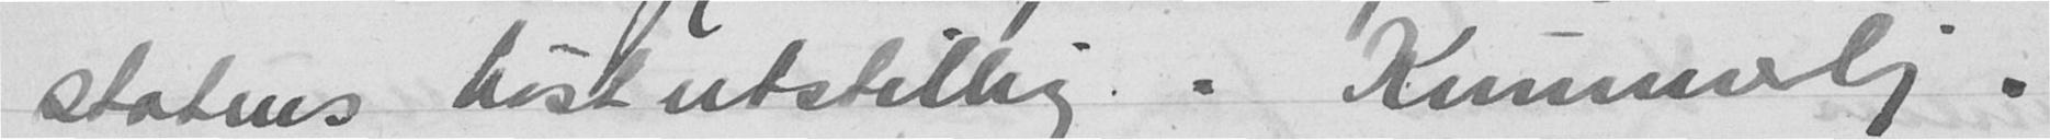statens høstutstillig. Kummerlig.
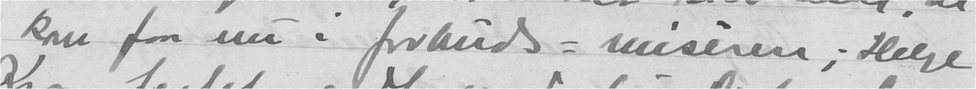kan faa nu i forbuds-misèren; Helge
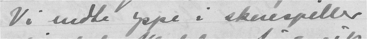Vi endte oppe i skuespiller
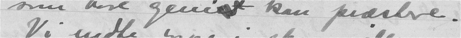som bare geniet kan præstere.
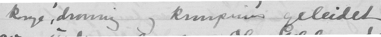konge, dronning og kronprins geleidet
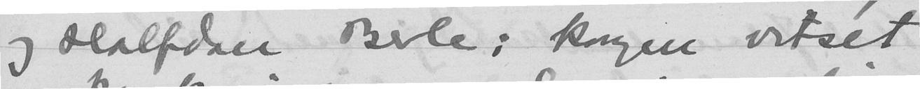og Halfdan Berle; kongen vitset
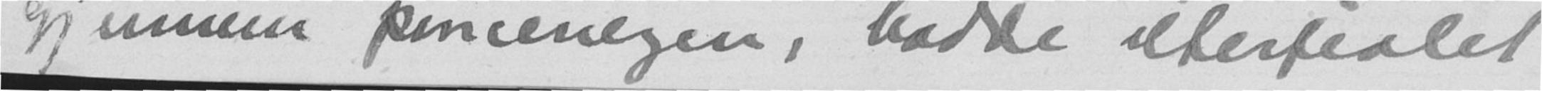gjennem pincenezen, hadde ilterfiolet
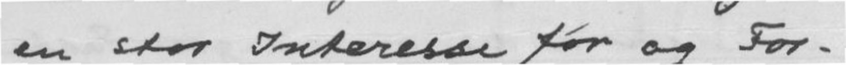en stor Interesse for og For-
